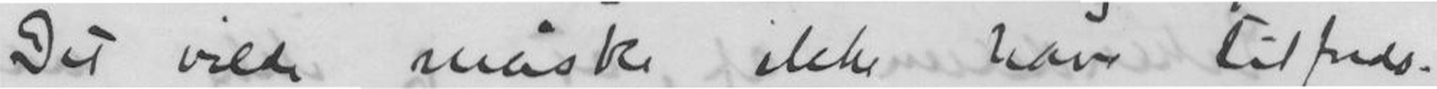Det vilde Måske ikke have tilfreds-
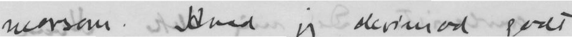morsom. Hvad jeg derimod godt
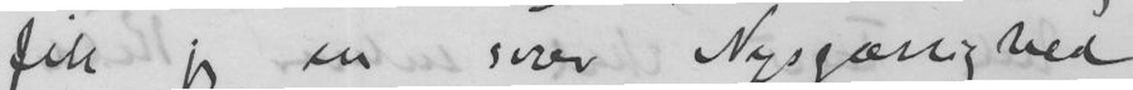fik jeg en svær Nysgærrighed
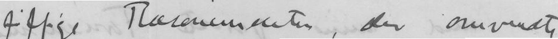fiffige Ræsonementer, der omvendte
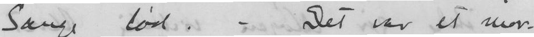Sange lød. Det var et mor-
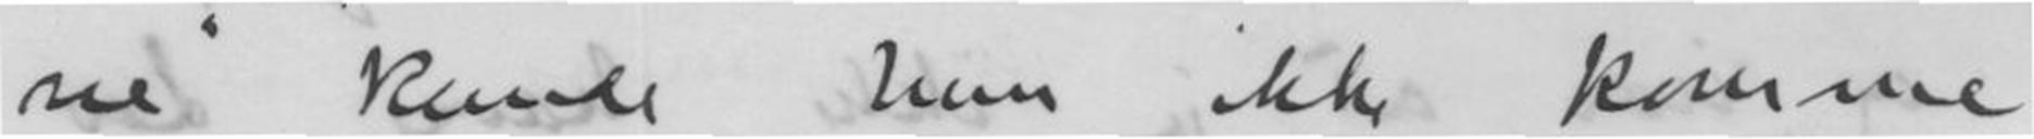ne kunde hun ikke komme
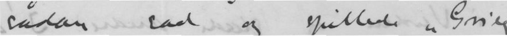sådan sad og spillede Grieg,
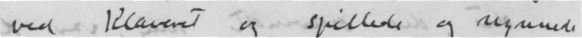ved Klaveret og spillede og nynnede.
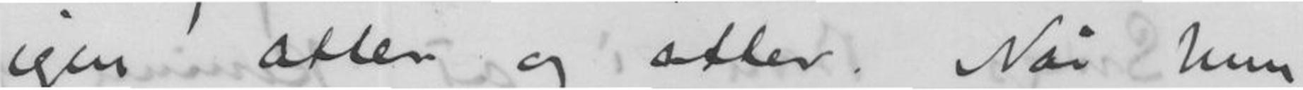igen atter og atter. Når hun
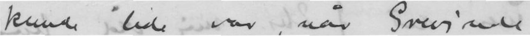kunde lide var, når Grevinde
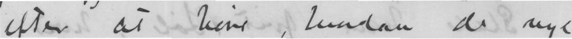efter at høre, hvordan de nye
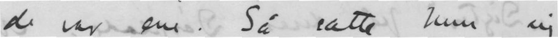de var ene. Så satte hun sig
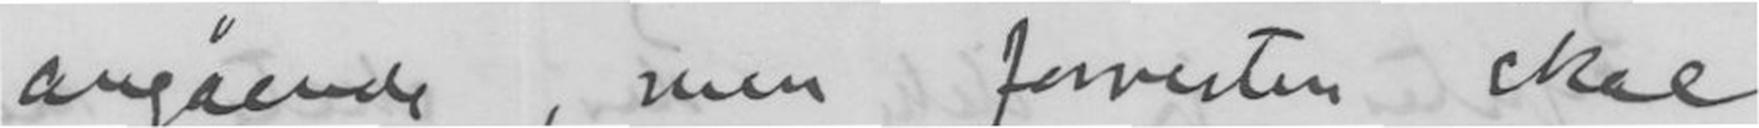angående, men foresten skal
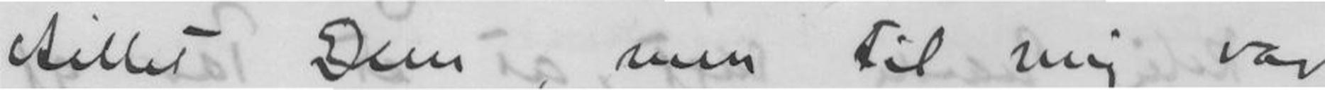stillet Dem, men til mig var
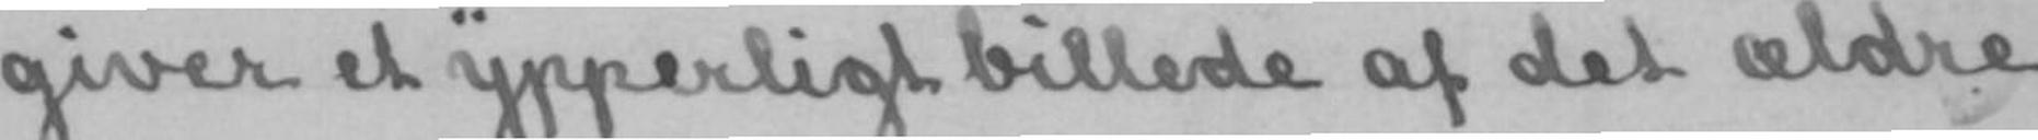giver et ypperligt billede af det ældre
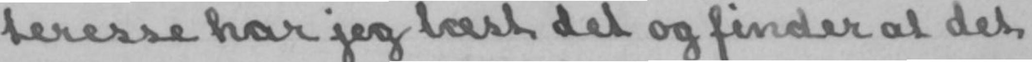teresse har jeg læst det og finder at det
